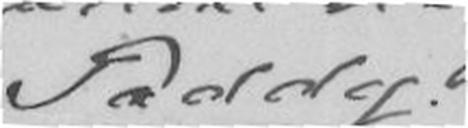Paddy.
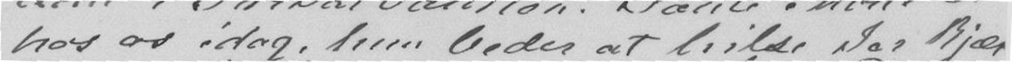hos os idag, hun beder at hilse Jer kjær-
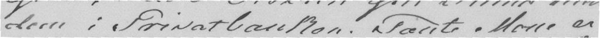dem i Privatbanken. Tante Mone er
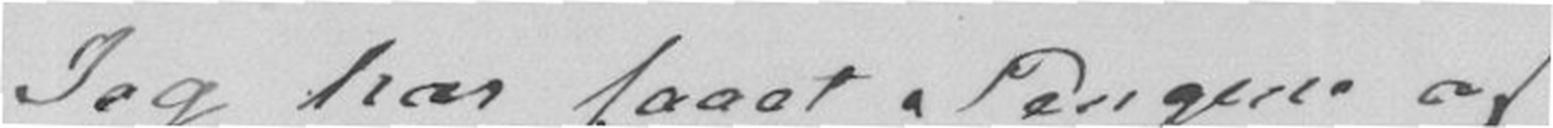Jeg har faaet Pengene af
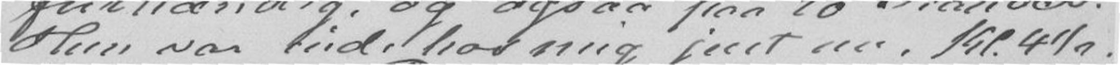Hun var inde hos mig just nu, Kl.4½.
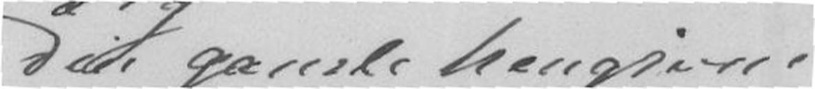Din gamle hengivne
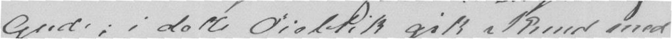Gude; i dette Øieblik gik Knud med
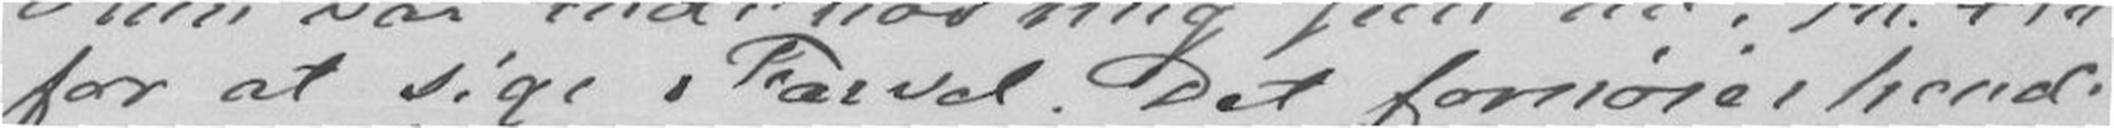for at sige Farvel. Det fornøier hende
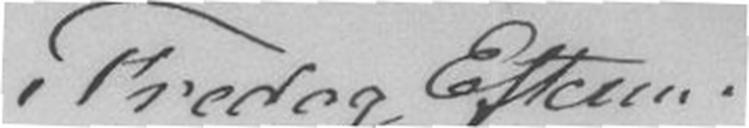Fredag Efterm.
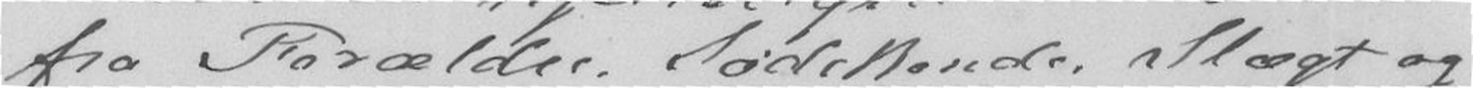fra Forældre, Sødskende, Slægt og
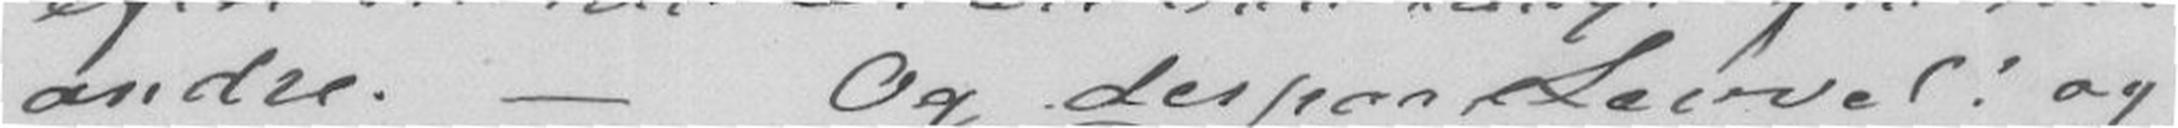andre. - Og derpaa Levvel! og
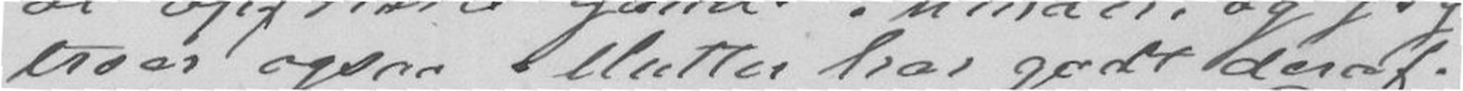troer ogsaa Mutter har godt deraf.
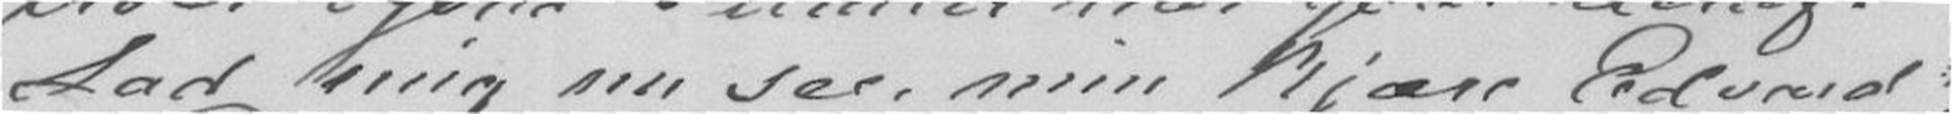Lad mig nu see, min kjære Edvard?
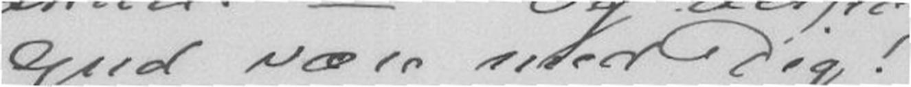Gud være med Dig!
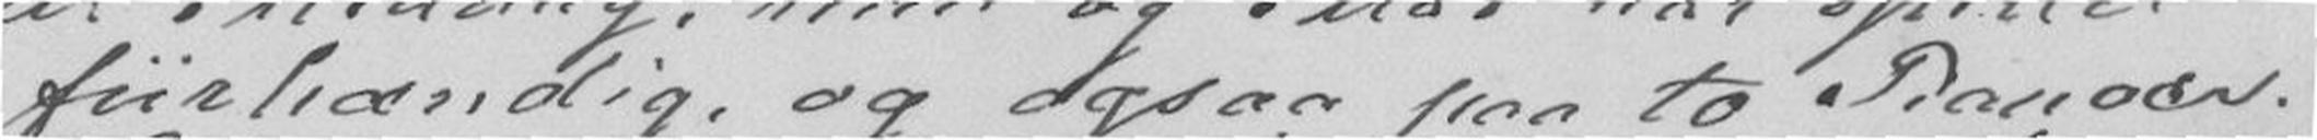fiirhændig, og ogsaa paa to Pianoer.
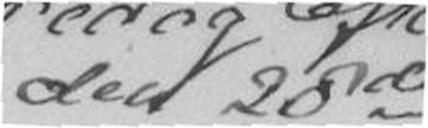den 28de
